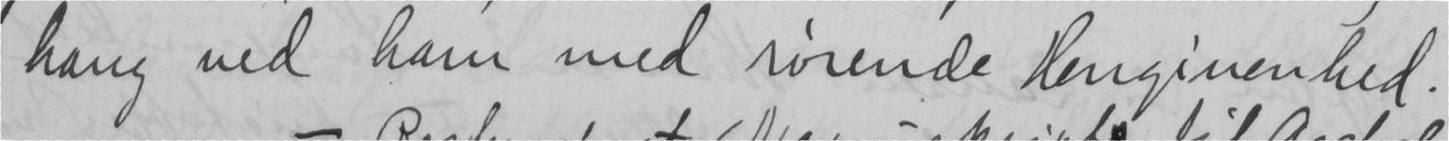hang ved ham med rørende Hengivenhed.
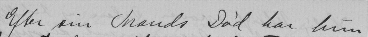Efter sin Mands Død har hun
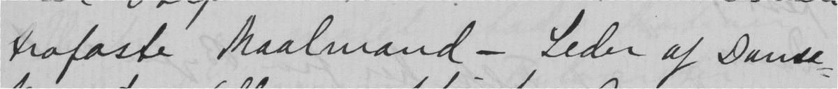trofaste maalmand - Leder af Danse-
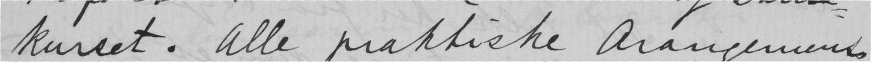kurset. Alle praktiske Arangements
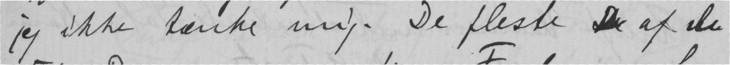jeg ikke tænke mig. De fleste  af de
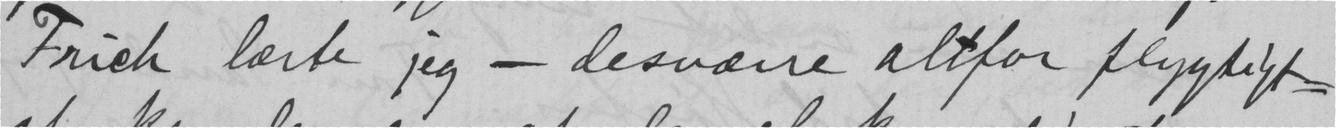Frich lærte jeg - desværre altfor flygtigt-
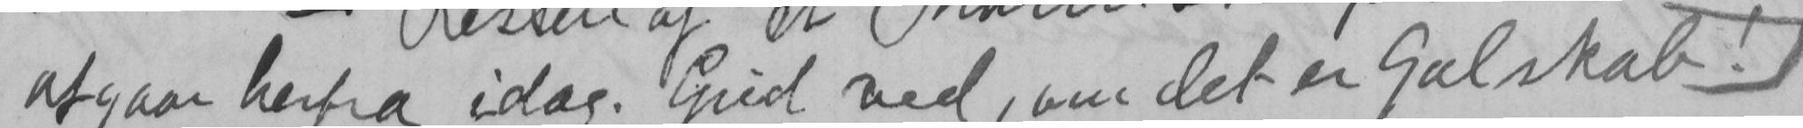Afgaar herfra idag. Gud ved, om det er Galskab!
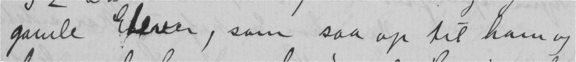gamle Elever, som saa op til ham og
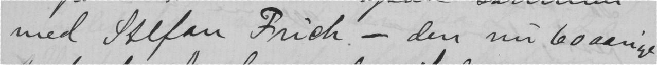med Stefan Frich - den nu 60 aarige
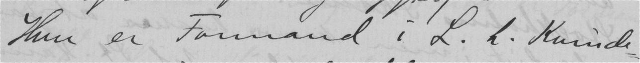Hun er Formand i L. h. Kvinde-
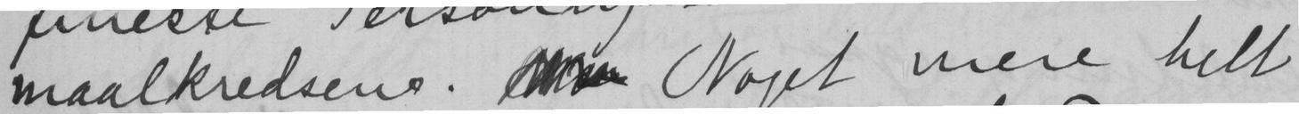maalkredsens.  Noget mere helt
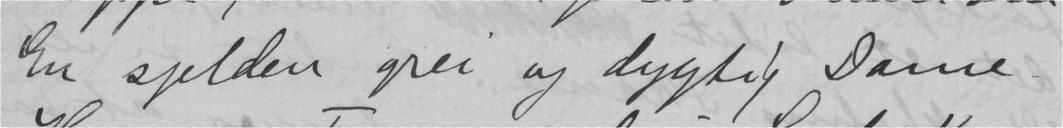En sjelden grei og dygtig Dame.
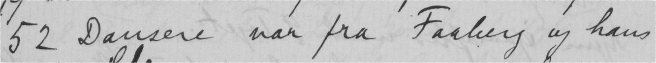52 Dansere var fra Faaberg og hans
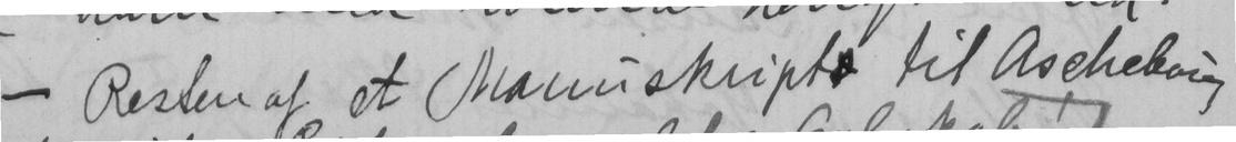- Resten af et Manuskript til Aschehoug
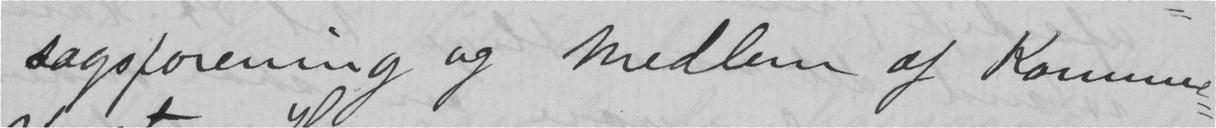sagsforening og medlem af Komune-
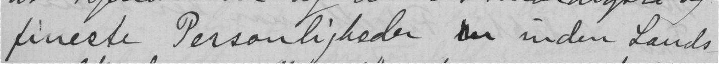fineste Personligheder  inden Lands
